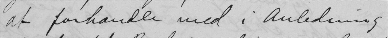at forhandle med i Anledning
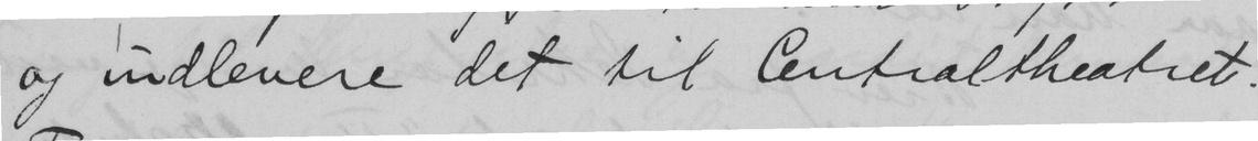og indlevere det til Centraltheatret.
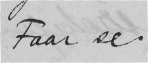Faar se.
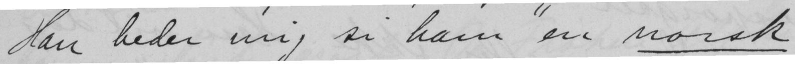Han beder mig si ham "en norsk
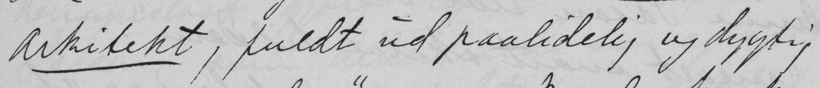arkitekt, fuldt ud paalidelig og dygtig
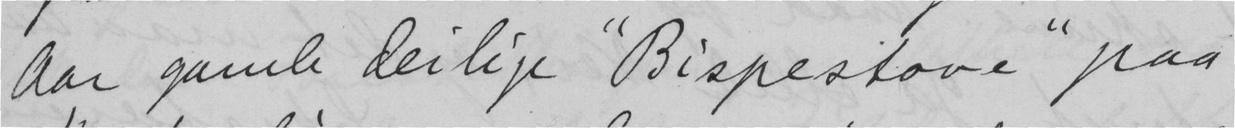Aar gamle deilige "Bispestove" paa
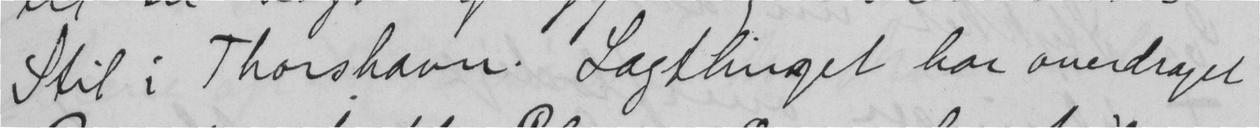Stil i Thorshavn. Lagthinget har overdraget
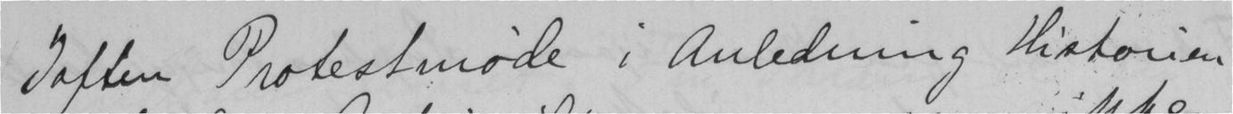Iaften Protestmøde i Anledning Historien
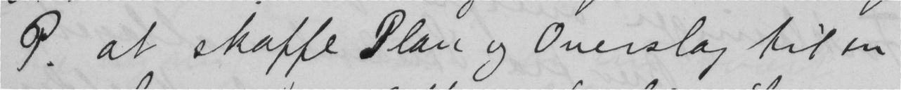P. at skaffe Plan og Overslag til en
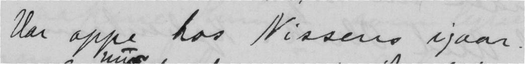Var oppe hos Nissens igaar.
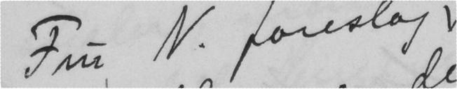Fru N. foreslog
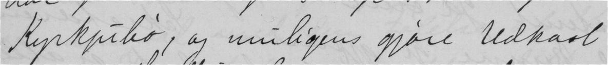Kyrkjubø, og muligens gjøre Udkast
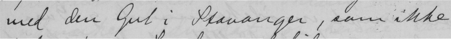med den Gut i Stavanger, som ikke
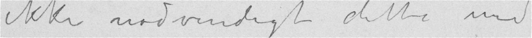ikke nødvendigt dette med
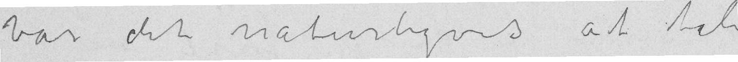var det naturligvis at tale
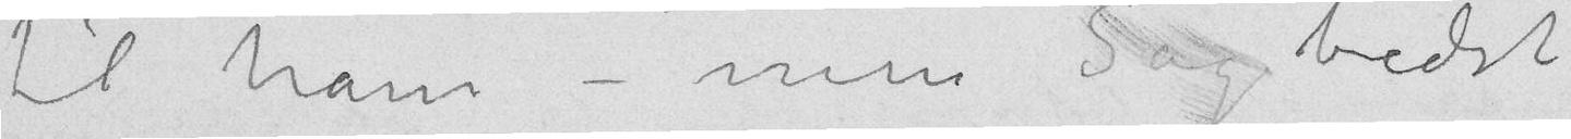til ham – men Sag bedst
